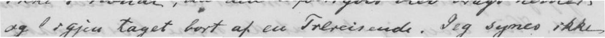og igjen taget bort af en Tilreisende. Jeg synes ikke
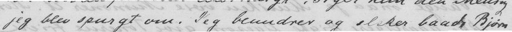jeg blev spurgt om. Jeg beundrer og elsker baade Bjørn-
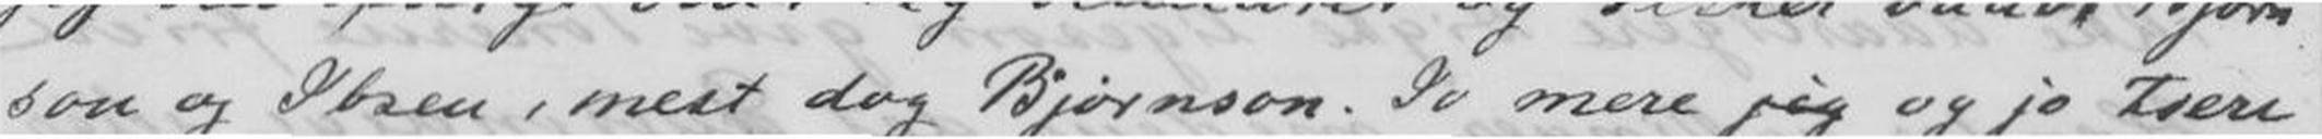son og Ibsen, mest dog Bjørnson. Jo mere jeg og jo tiere
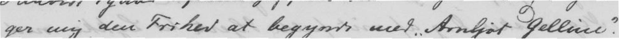ger mig den Frihed at begynde med Arnljot Galline.
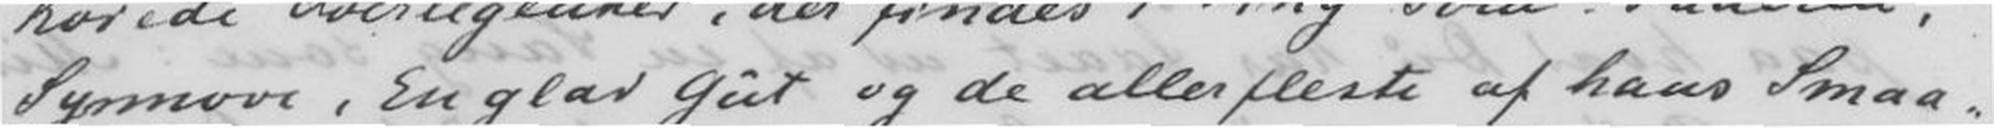Synnøve, Englad Gut og de allerfleste af hans Smaa-
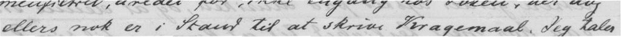ellers nok er i Staand til at skrive Kragemaal. Jeg taler
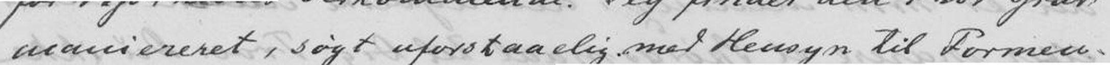maniereret, søgt uforstaaelig med Hensyn til Formen.
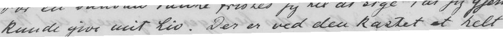kunde give mit Liv. Der er ved den kastet et helt
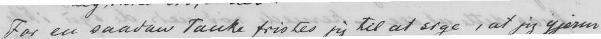For en saadan Tanke fristes jeg til at sige, at jeg gjerne
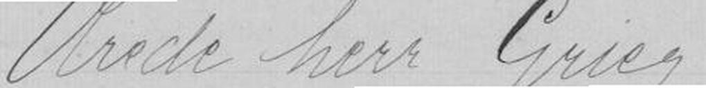Ærede herr Grieg!
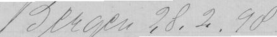Bergen 28.2.98
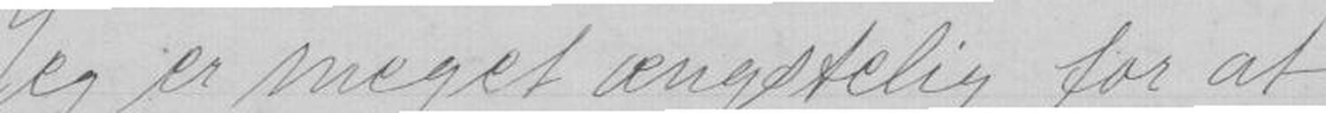Jeg er meget ængstelig for at
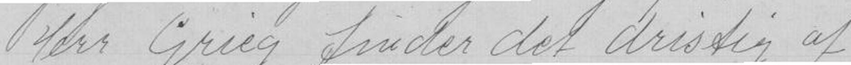herr Grieg finder det dristig af
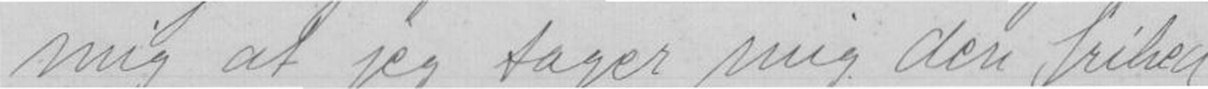mig at jeg tager mig den frihed
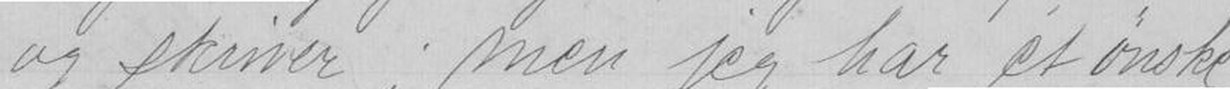og skriver, men jeg har et önske
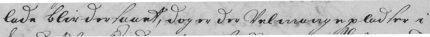lade blir der saaet, dog er der Vel mange pladser i
